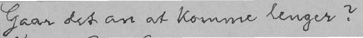Gaar det an at komme lenger?
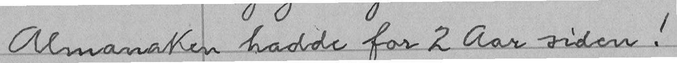Almanaken hadde for 2 Aar siden!
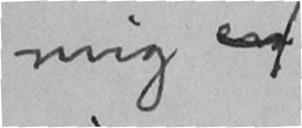mig en
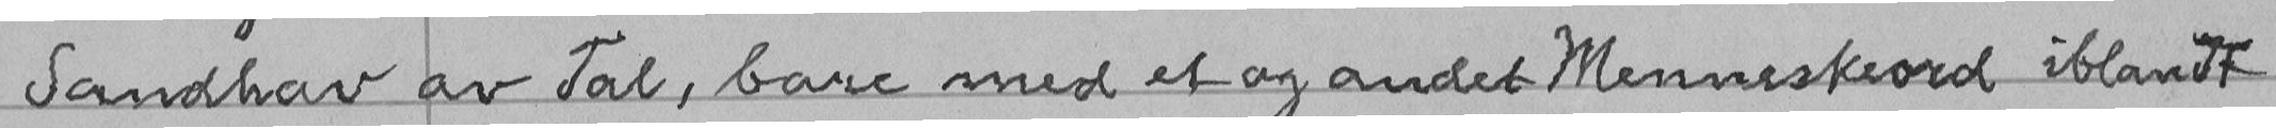Sandhav av Tal, bare med et og andet Menneskeord iblandt
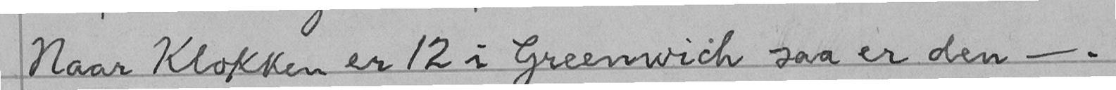Naar Klokken er 12 i Greenwich saa er den -.
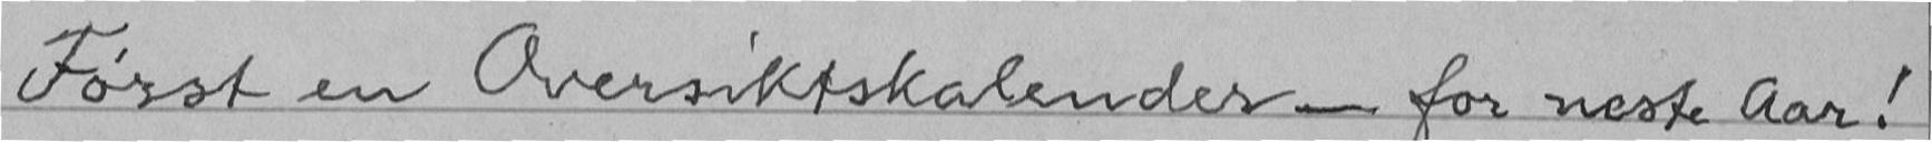Først en Oversiktskalender - for neste Aar!
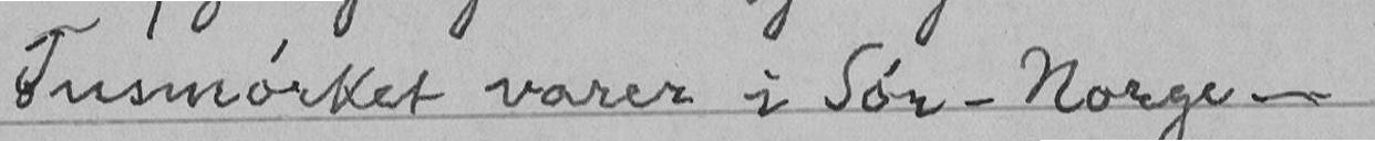Tusmørket varer i Sør-Norge -.
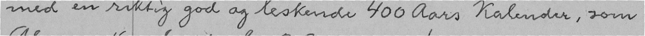med en riktig god og leskende 400 Aars Kalender, som
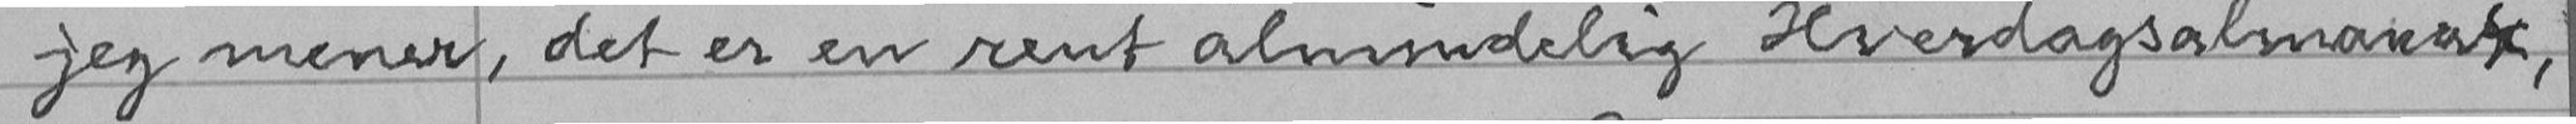jeg mener, det er en rent almindelig Hverdagsalmanak,
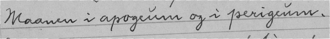Maanen i apogeum og i perigeum.
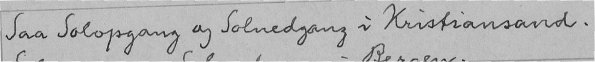Saa Solopgang og Solnedgang i Kristiansand.
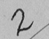2
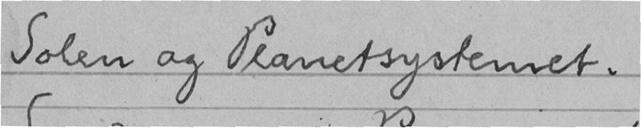Solen og Planetsystemet.
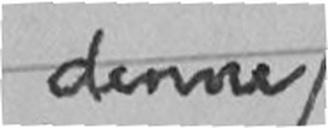denne
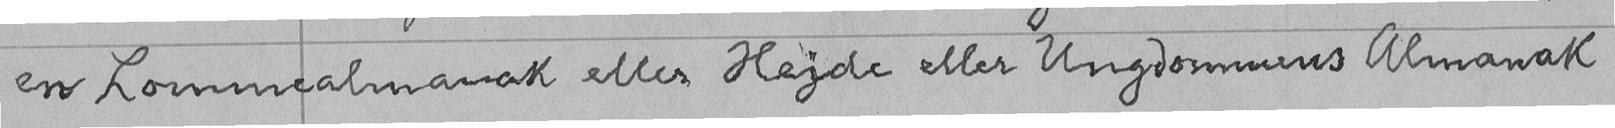en Lommealmanak eller Hejde eller Ungdommens Almanak
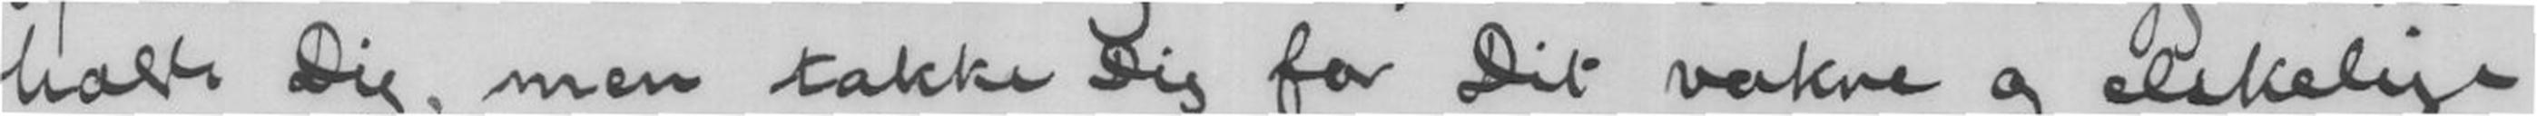holde Dig, men takke Dig for Dit vakre og elskelige
